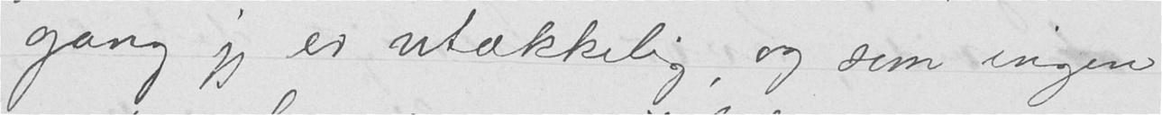gang jeg er utækkelig, og som ingen
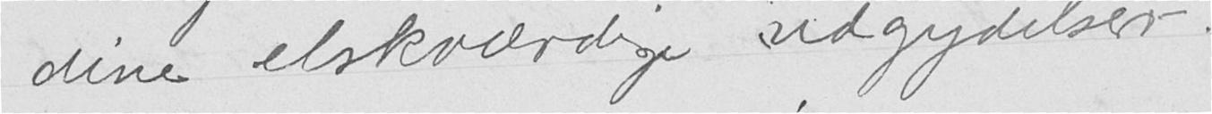dine elskværdige udgydelser.
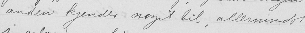anden kjender noget til, allermindst
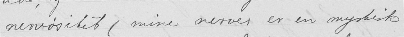nervøsitet (mine nerver er en mystisk
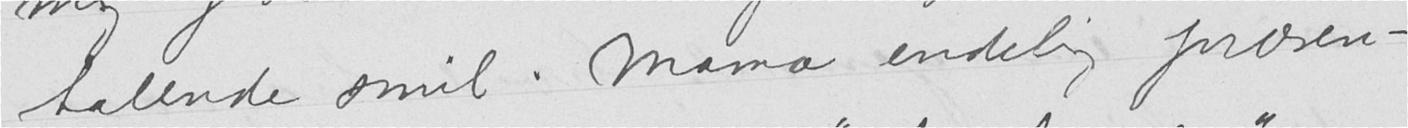talende smil. Mama endelig præsen-
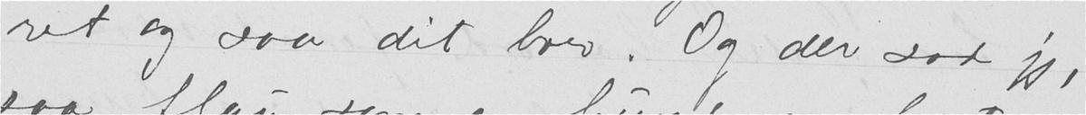ret og saa dit brev. Og der sad jeg,
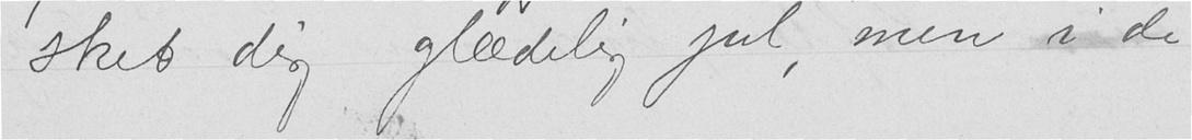sket dig glædelig jul, men i de
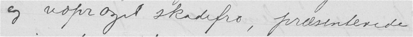og udpræget skadefro, præsenterede
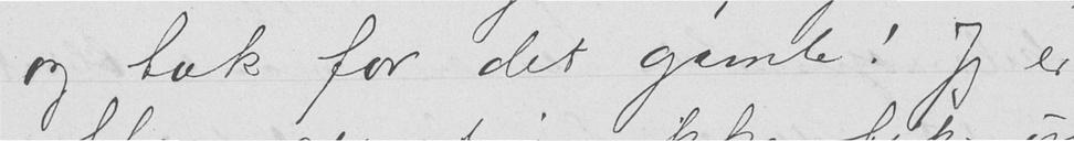og tak for det gamle! Jeg er
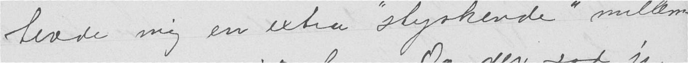terede mig en extra "styrkende" mellem-
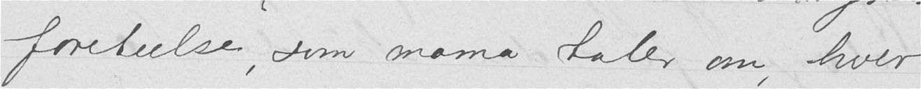foreteelse, som mama taler om, hver
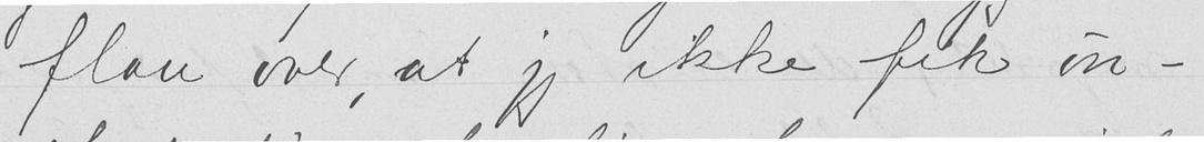flau over, at jeg ikke fik øn-
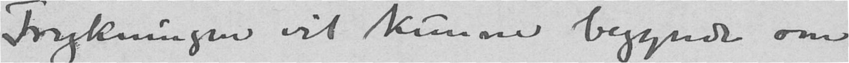Trykningen vil kunne begynde om
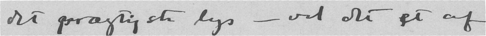det prægtigste lys - vel et af
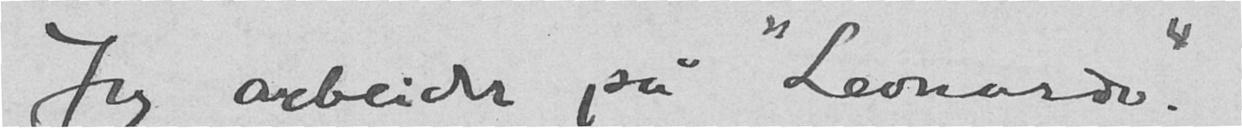Jeg arbeider på "Leonardo".
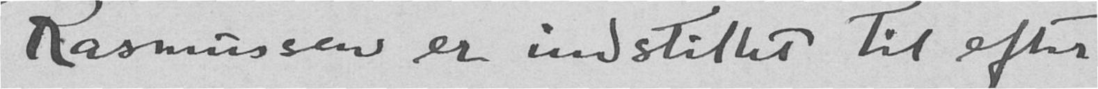Rasmussen er indstillet til efter
